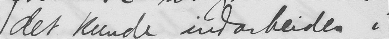det kunde indarbeides i
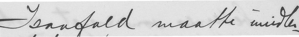Isaafald maatte imidler-
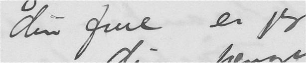din frue er jeg
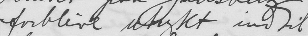forblive utrykt indtil
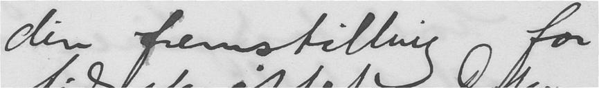din fremstilling for
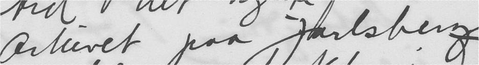arkivet paa Jarlsberg
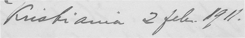Kristiania 2 febr. 1911.
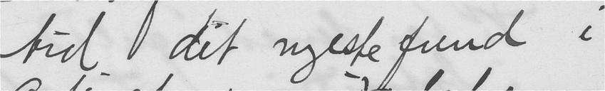tid dit nyeste fund i
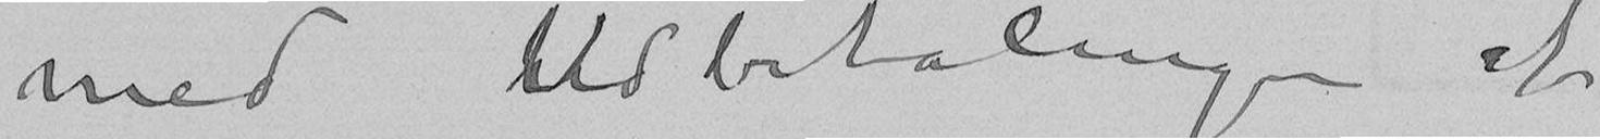med Udbetalingen af
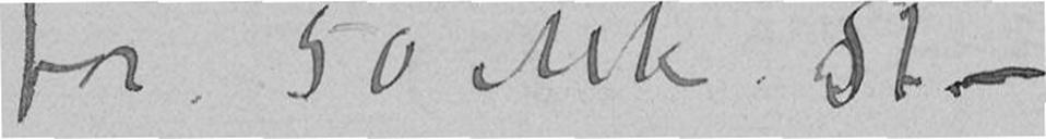for 50 Mk St –
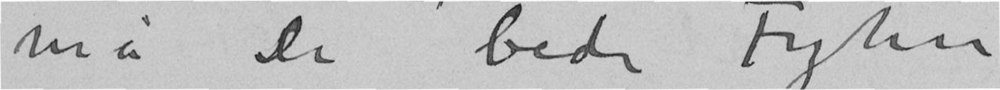må De bede Fyhn
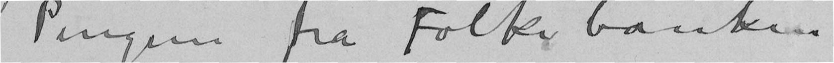Pengene fra Folkebanken
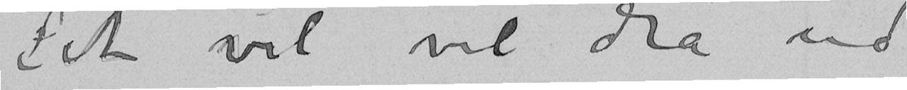Det vil vel dra ud
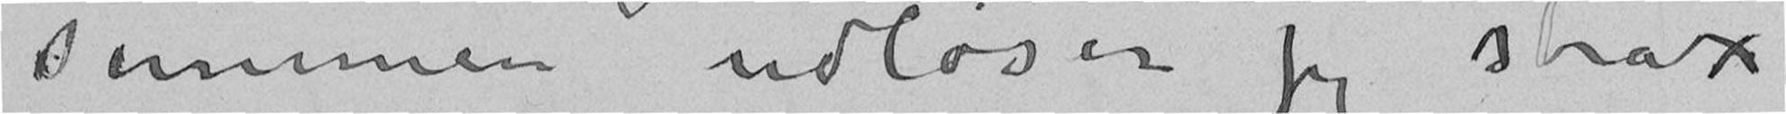summen udløser jeg strax
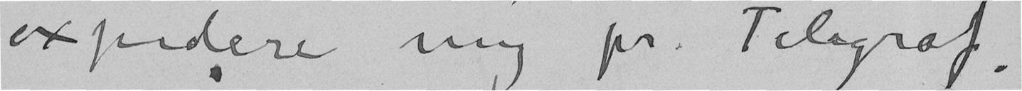expedere mig pr. Telegraf.
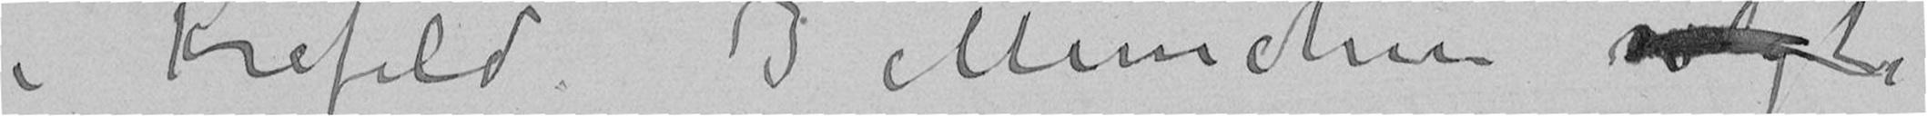i Krefeld. I München solgte
